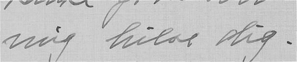mig hilse dig.
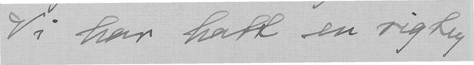Vi har hatt en rigtig
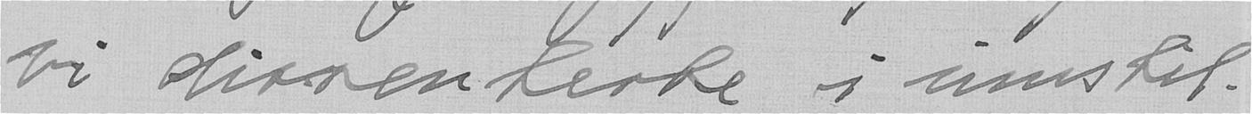vi dissenterte i instil-
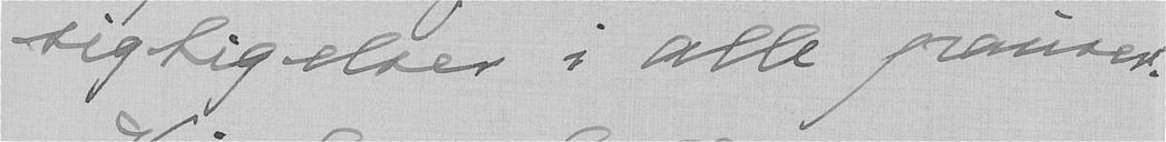sigtigelser i alle pauser.
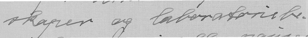skaper og laboratoriebe-
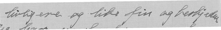luvligere og like fin og beskjeden.
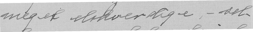meget elskverdige, - sel-
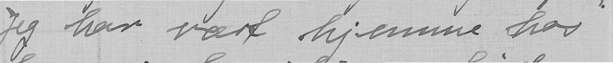Jeg har vært hjemme hos
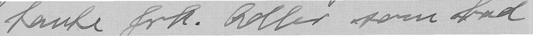tante frk. Adler som bad
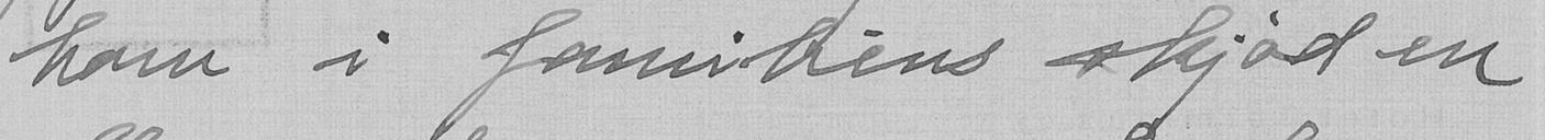ham i familiens skjød en
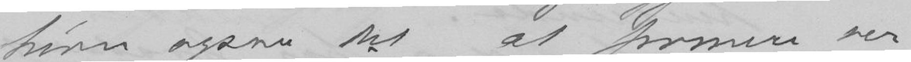høres ogsaa det at, formere ver-
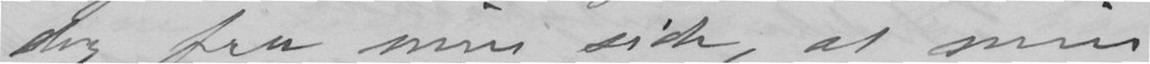dog fra min side, at min
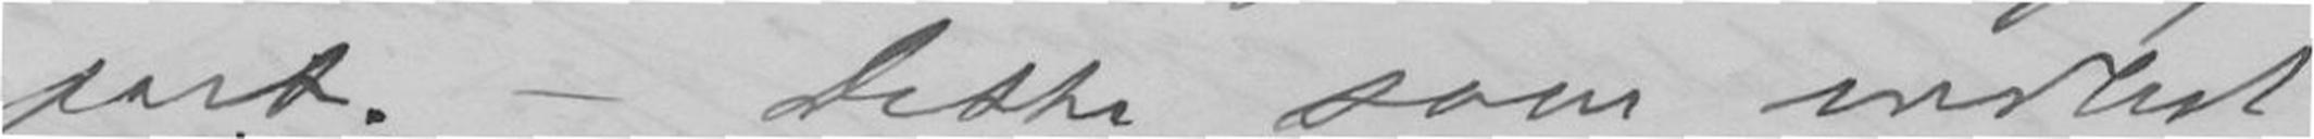part. Dette som indled-
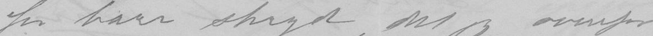for bare skryd, det jeg ovenfor
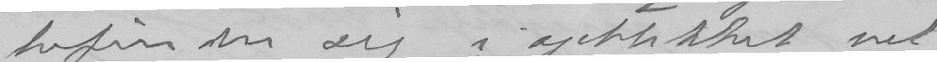befinder sig i øjeblikket vel.
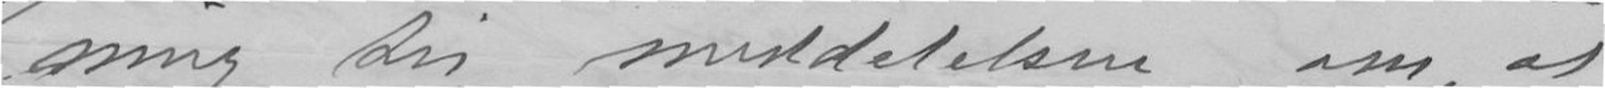ning til meddelsen om, at
